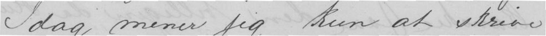Idag mener jeg kun at skrive
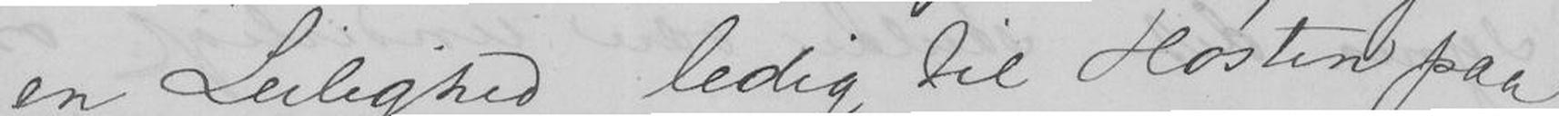en Leilighed ledig til Høsten paa
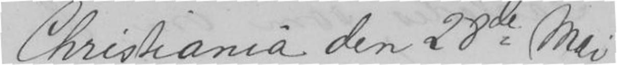Christiania den 28de Mai.
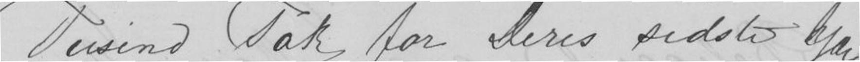Tusind Tak for Deres sidste kjære
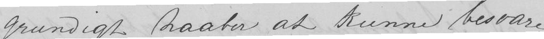grundigt haaber at kunne besvare.
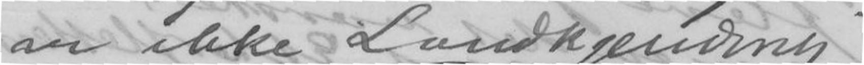vi ikke "Landkjending"!!
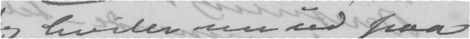Jeg hviler nu ud paa
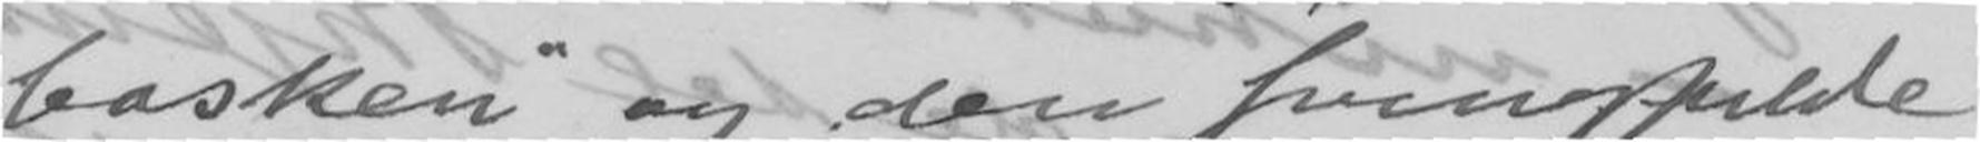basken" og den svingfulde
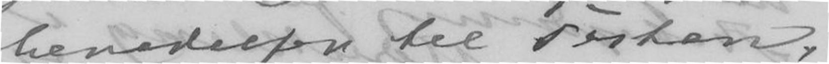beredelser til Festen,
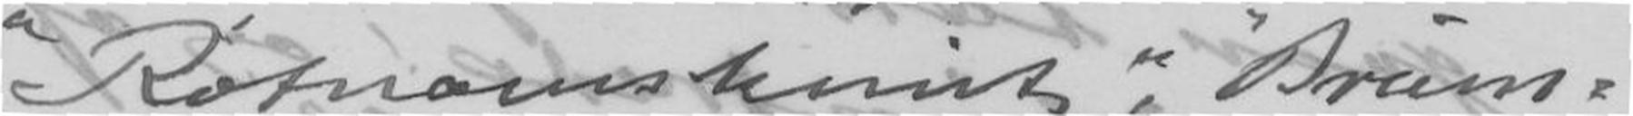"Røtnamsknut", "Brum-
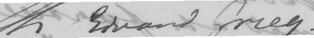Hr Edvard Grieg.
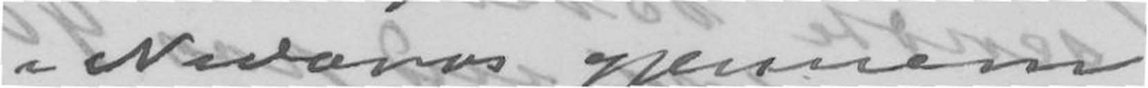i Nidaros gjennem
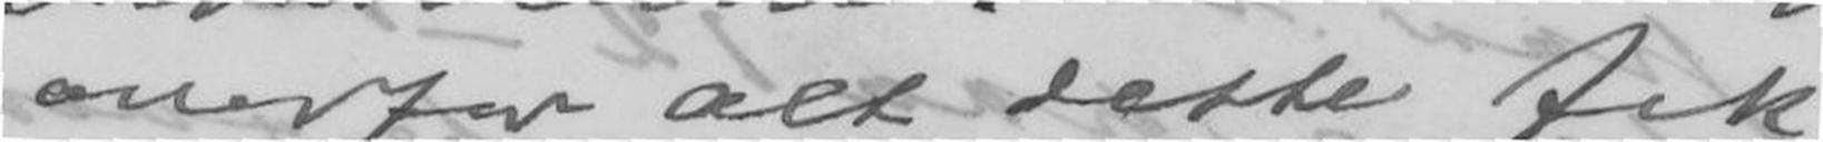overfor alt dette fik
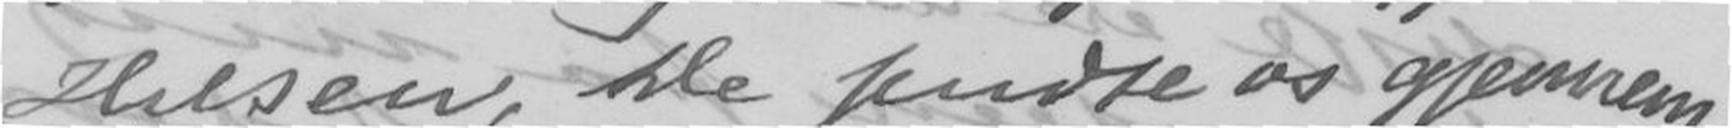Hilsen, De sende os gjennem
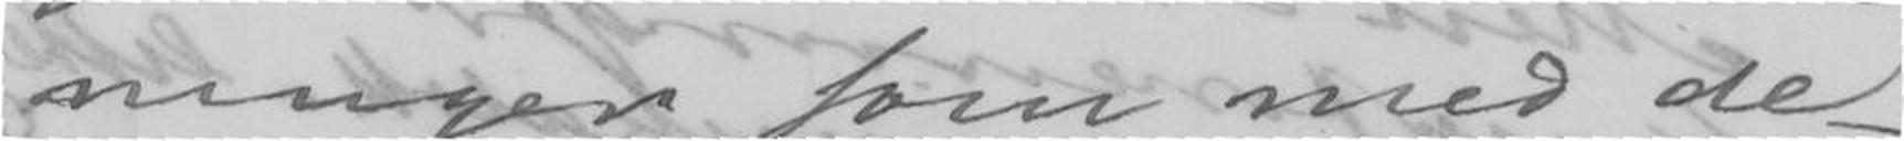ninger som med de
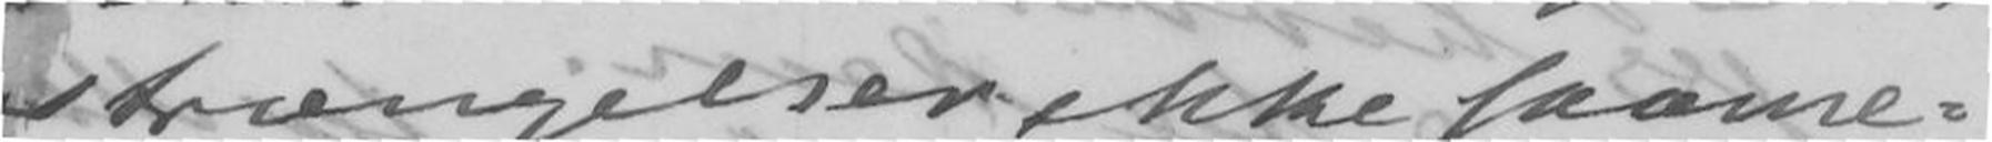strængelser ikke saame-
